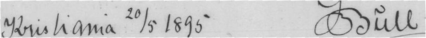Kristiania 20/5 1895 JBBull
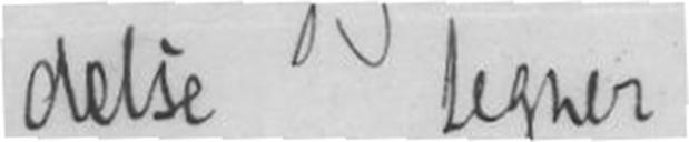delse tegner
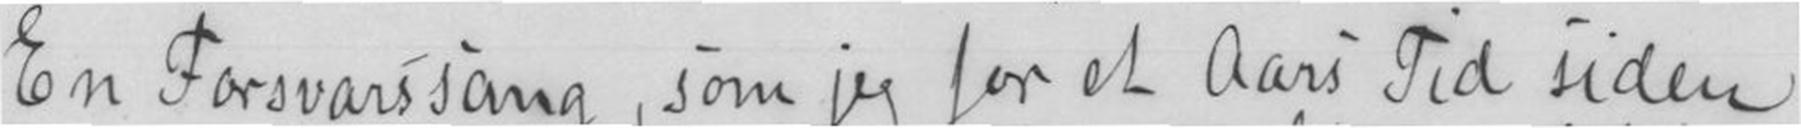En Forsvarssang, som jeg for et Aars Tid siden
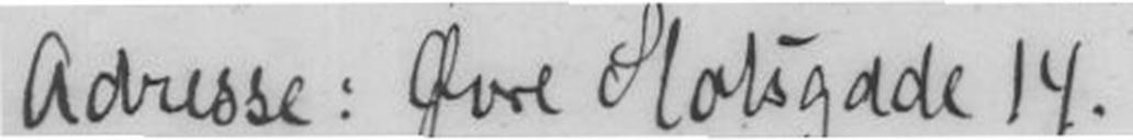Adresse: Øvre Stølsgade 14.
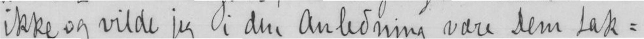ikke og vilde jeg i den Anledning være Dem tak-
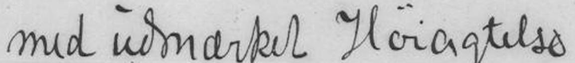med udmærket Høiagtelse
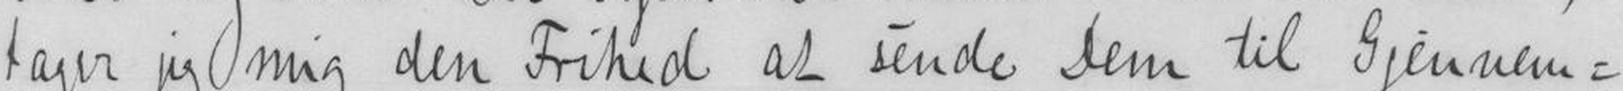tager jeg mig den Frihed at sende Dem til Gjennem-
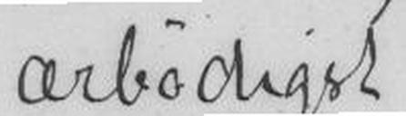ærbødigst
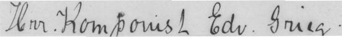Hrr. Komponist Edv. Grieg.
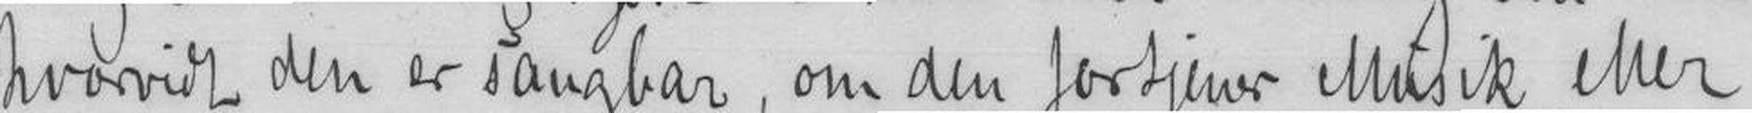hvorvidt den er sangbar, om den fortjener Musik eller
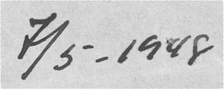7/5-1948
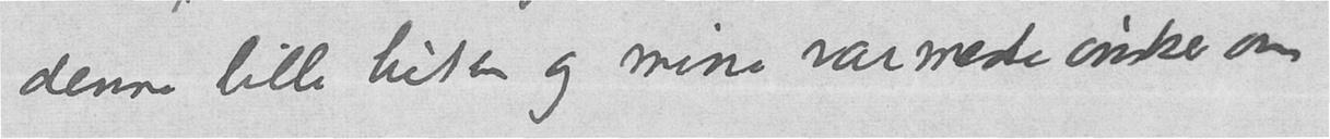denne lille hilsen og mine varmeste ønsker om
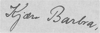Kjære Barbra,
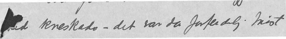med kneskade – det var da forferdelig trist
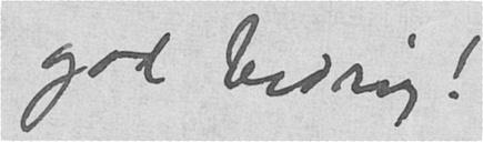god bedring!
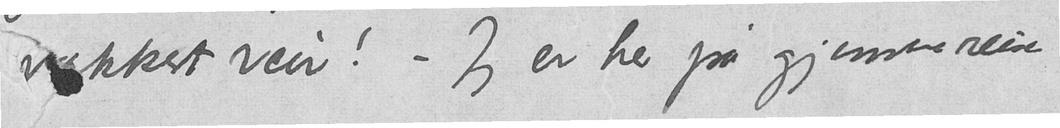vakkert veir! - Jeg er her på gjennemreise
